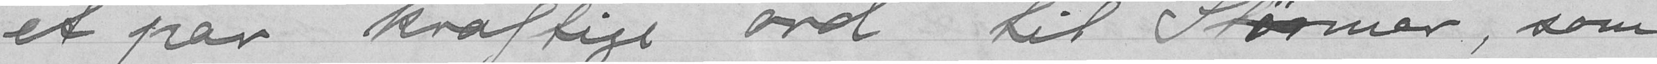et par kraftige ord til Størmer, som
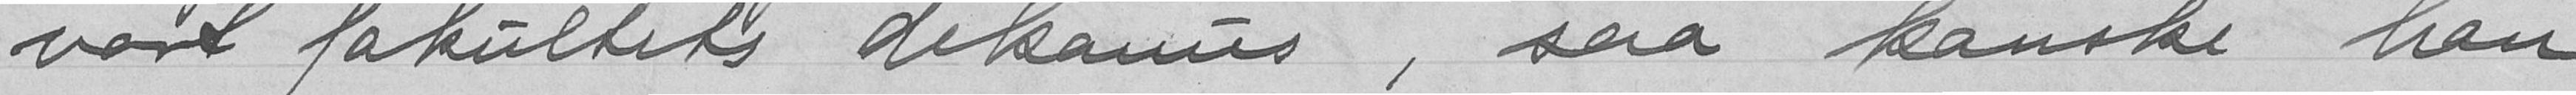vort fakultets dekanus, saa kanske han
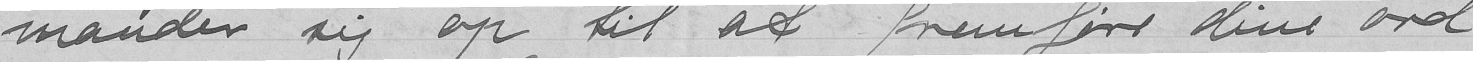mander sig op til at fremføre dine ord
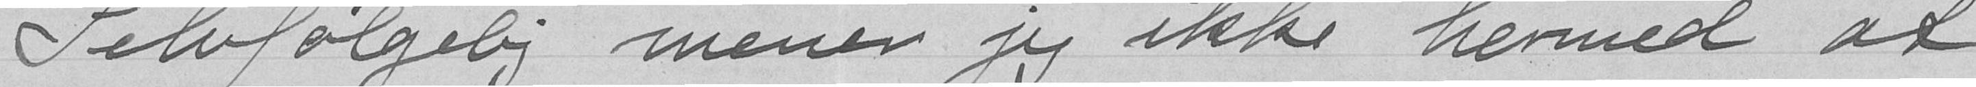Selvfølgelig mener jeg ikke hermed at
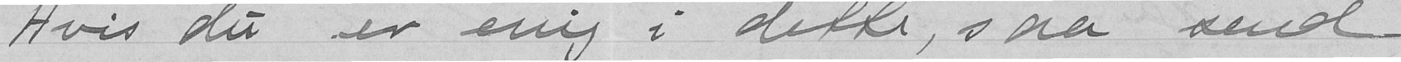Hvis du er enig i dette, saa send
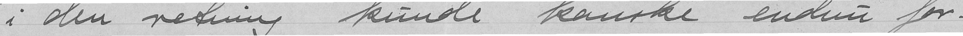i den retning kunde kanske endnu for-
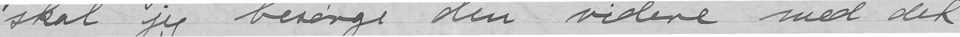skal jeg besørge den videre med det
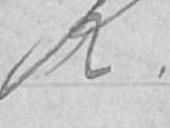K.
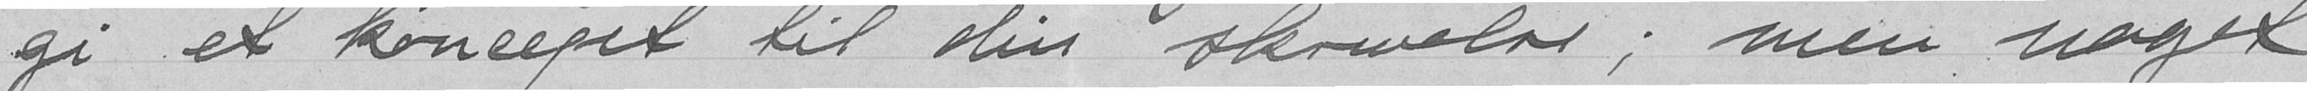gi et koncept til din skrivelse; men noget
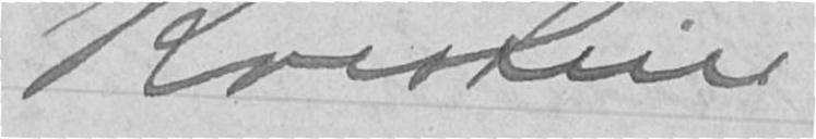Kristine
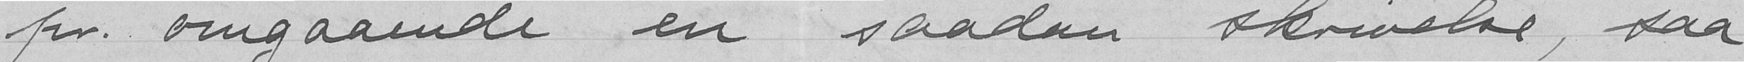for omgaaende en saadan skrivelse, saa
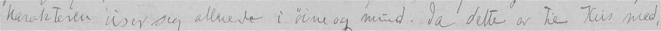karakteren viser sig allerede i øine og mund. Ja dette er til Kris med,
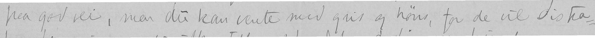paa god vei, men du kan vente med gris og høns, for de vil distra-
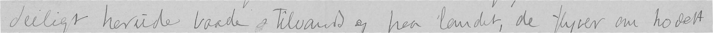deiligt herude baade  tilvands og paa landet, de flyver om hodet
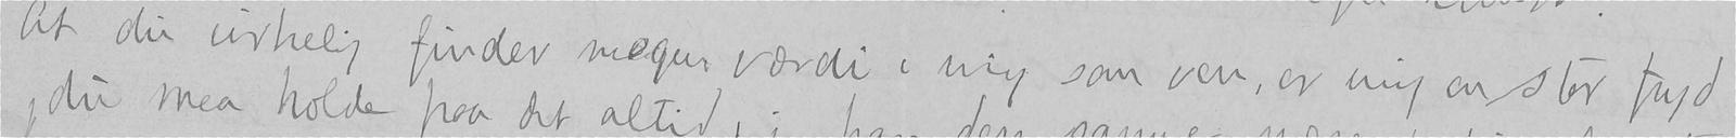At du virkelig finder megen værdi i mig som ven, er mig en stor fryd
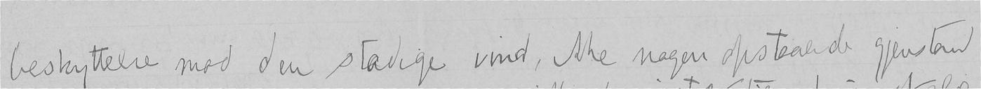beskyttelse mod den stadige vind, ikke nogen opstaaende gjenstand
